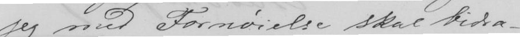jeg med Fornøielse skal bidra-
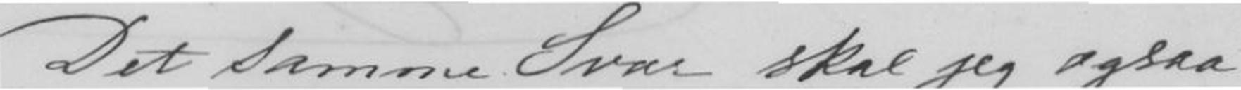Det samme Svar skal jeg ogsaa
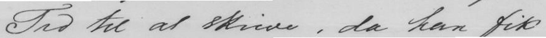Tid til at skrive, da han fik
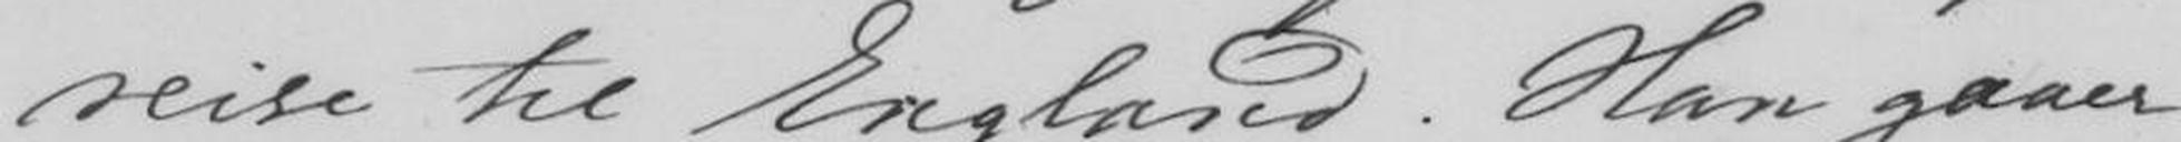reise til England. Han gaaer
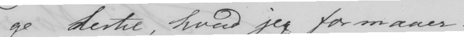ge dertil, hvad jeg formaar.
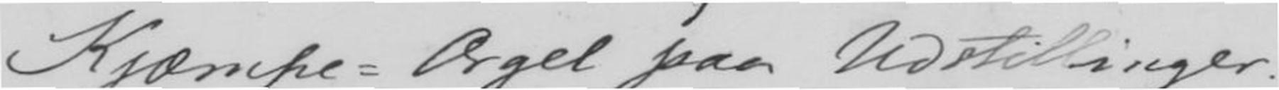Kjæmpe=Orgel paa Udstillinger.
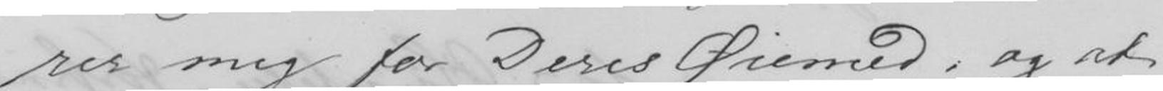rer mig for Deres Øiemed, og at
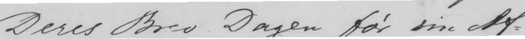Deres Brev Dagen før sin Af-
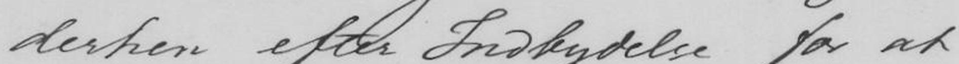derhen efter Indbydelse for at
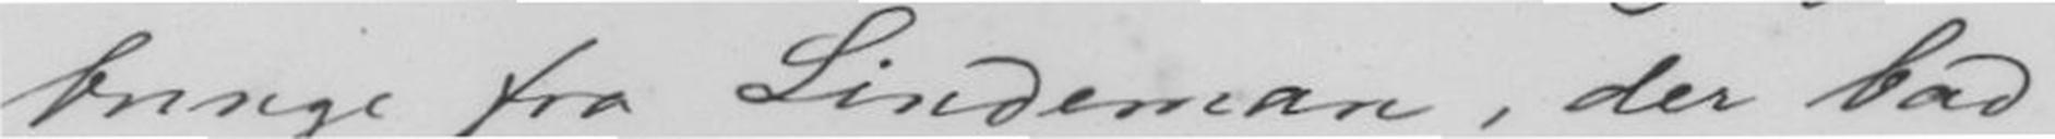bringe fra Lindeman, der bad
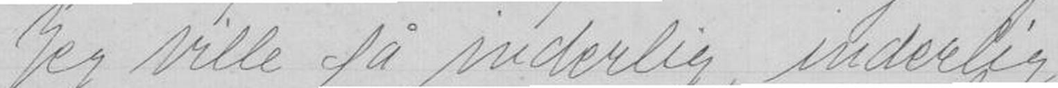-Jeg ville så inderlig, inderlig
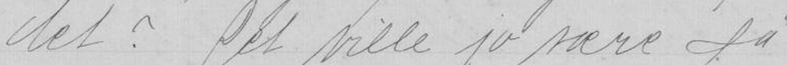det? Det ville jo være så
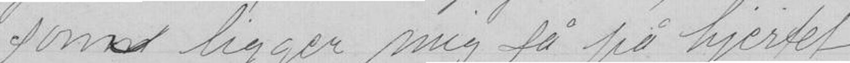som ligger mig så på hjertet
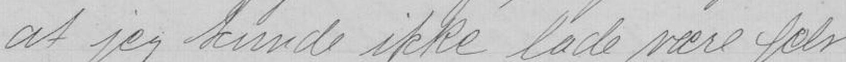at jeg kunde ikke lade være selv
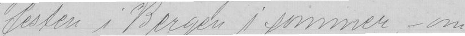festen i Bergen i sommer,- om
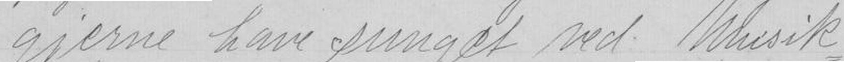gjerne have sunget ved Musik-
